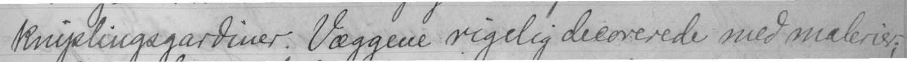kniplingsgardiner. Væggene rigelig decorerede med malerier;
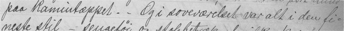paa kamintæppet. - Og i soveværelset var alt i den fi-
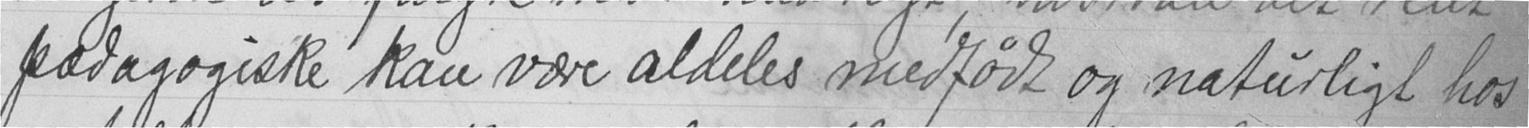pædagogiske kan være aldeles medfødt og naturligt hos
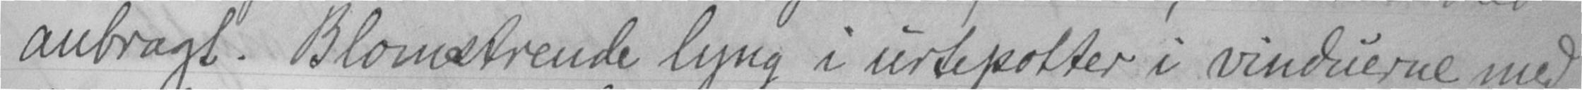anbragt. Blomstrende lyng i urtepotter i vinduerne med
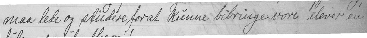maa lede og studere for at kunne bibringe vore elever en
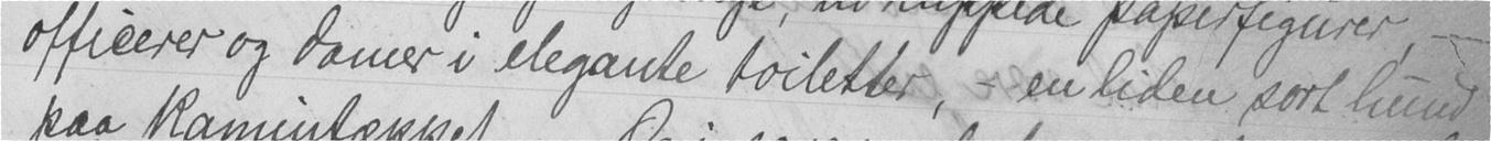officerer og damer i elegante toiletter, - en liden sort hund
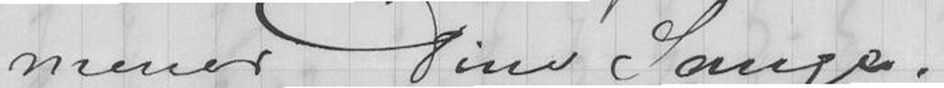mener Dine Sange. -
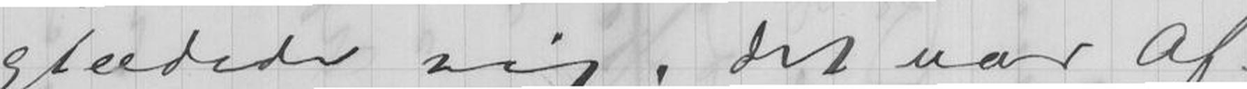glædede sig, Det var af-
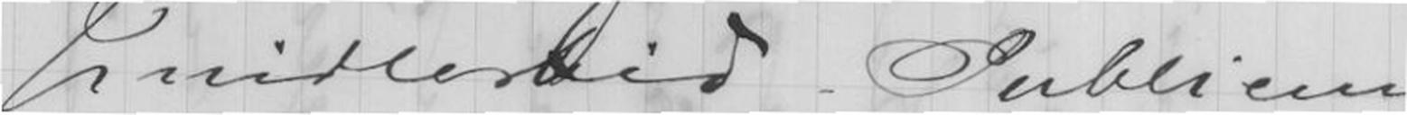Imidlertid Publicum
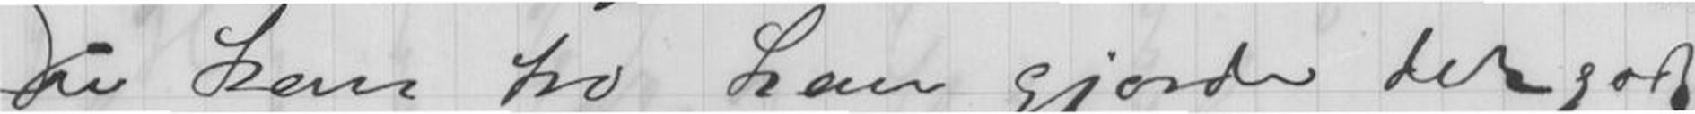Du kan tro han gjorde det godt
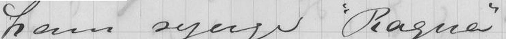ham synge "Ragna" -
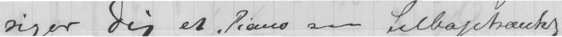siger Dig et Piano saa tilbagetrænkt
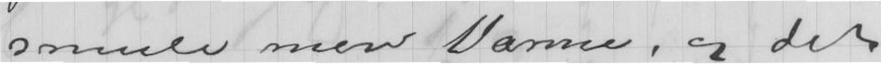smule mere Varme, og det
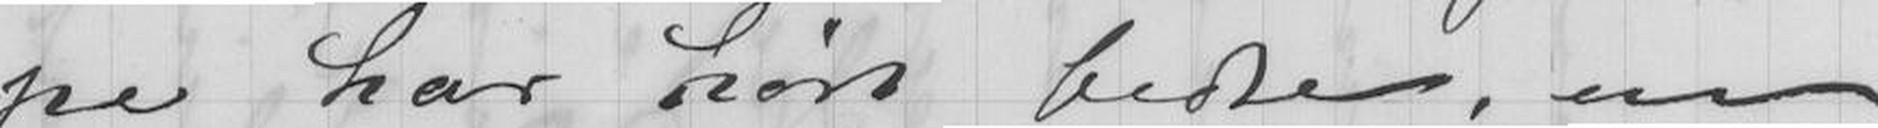pe har hørt bedre, en
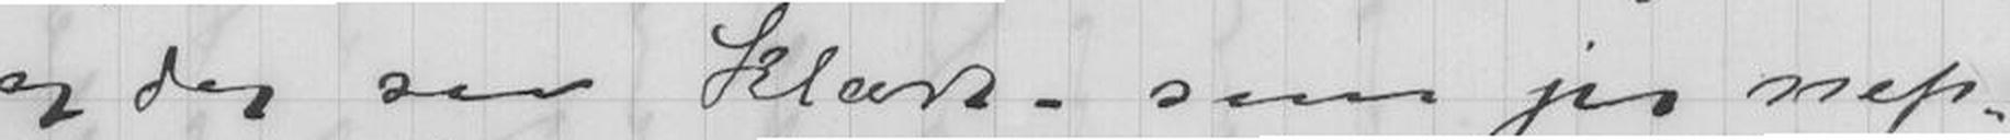og dog saa klart. som jeg nep-
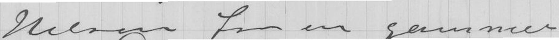Hilsen fra en gammel
